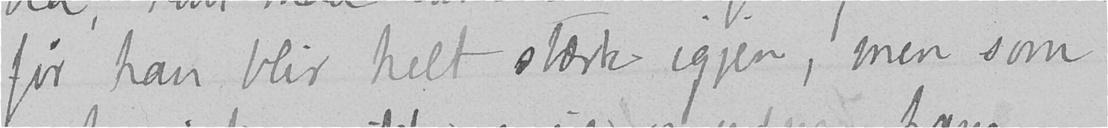før han blir helt stærk igjen, men som
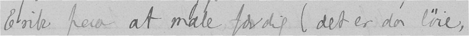Erik paa at male færdig (det er da løie,
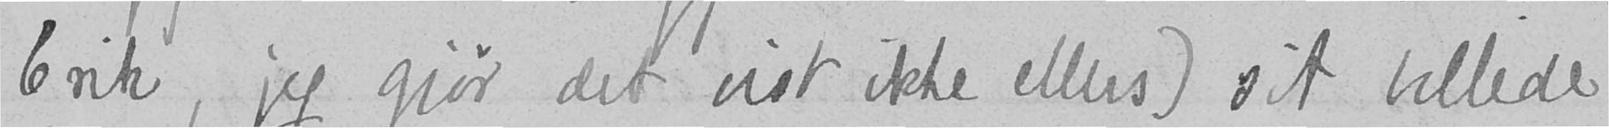Erik, jeg gjør det vist ikke ellers) sit billede
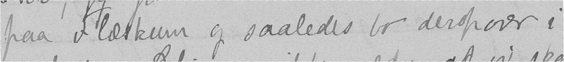paa Flæskum og saaledes bo deropover i
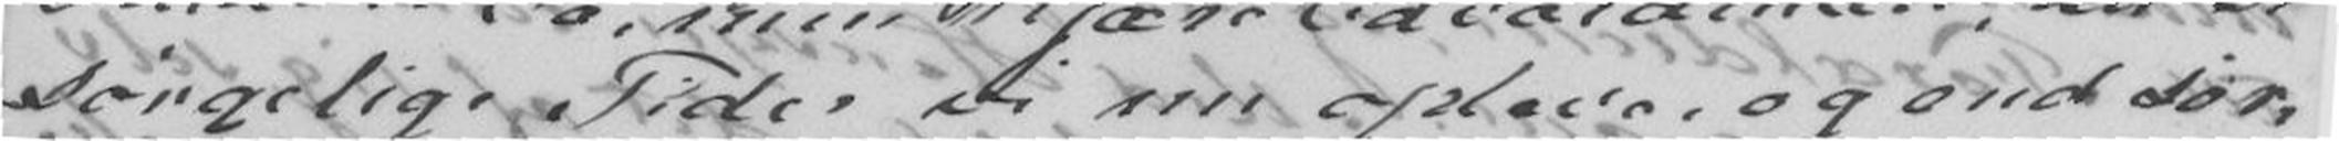sørgelige Tider vi nu opleve, og end sør-
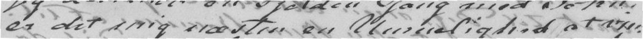er det mig næsten en Umuelighed at vin-
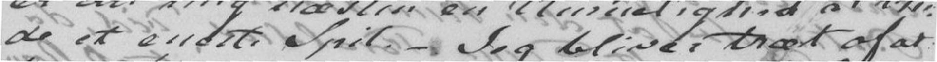de et eneste Spil. - Jeg bliver træt af at
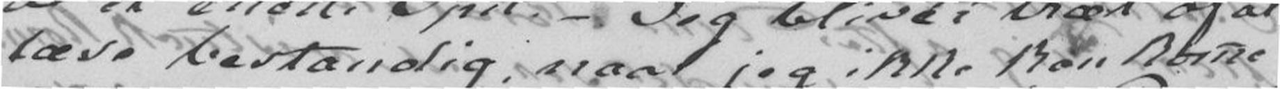læse bestandig, naar jeg ikke kan ko`me
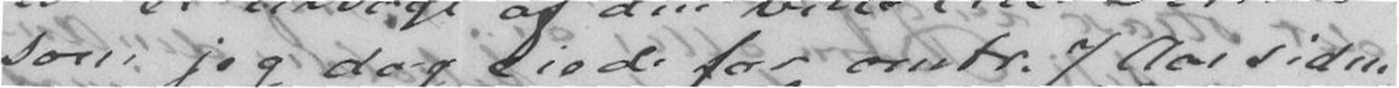som jeg dog eiede for omtr. 7 Aar siden,
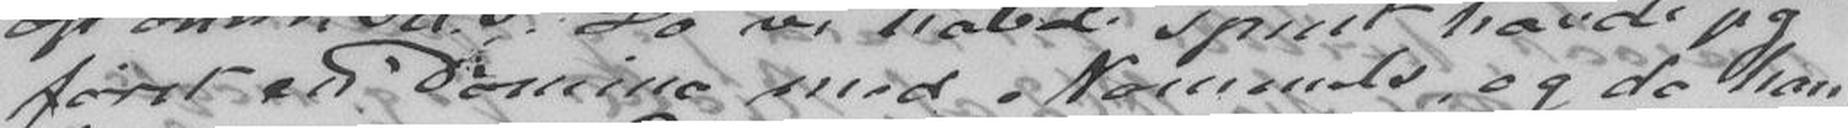først en Domino med Nommels, og da han
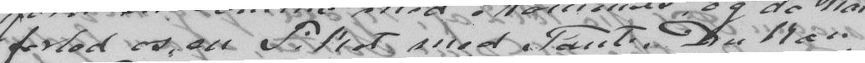forlod os, en Pikot med Tante. Du kan
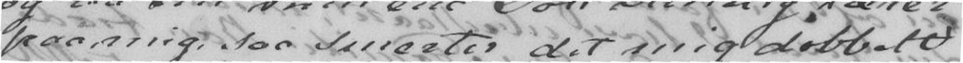paa mig, saa smerter det mig dobbelt
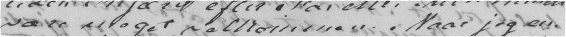være meget velkommen. Naar jeg en-
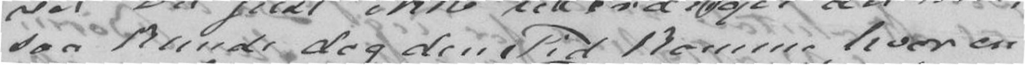saa kunde dog den Tid komme hvor en
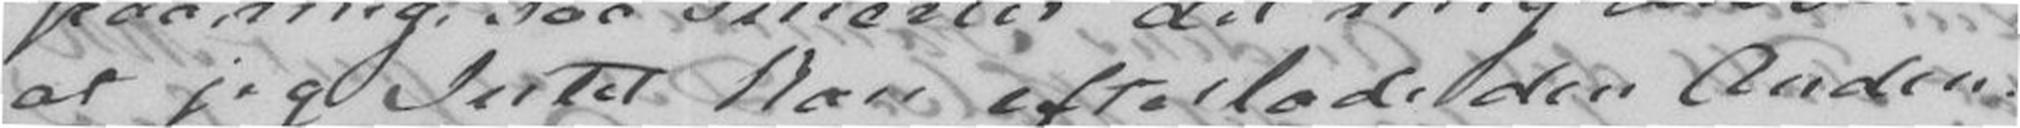at jeg Intet kan efterlade den Anden.
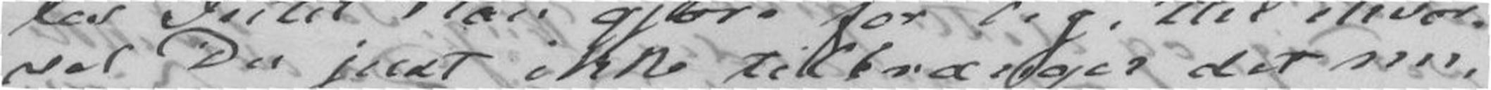vel Du just ikke tiltrænger det nu,
